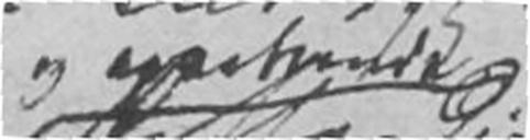og X
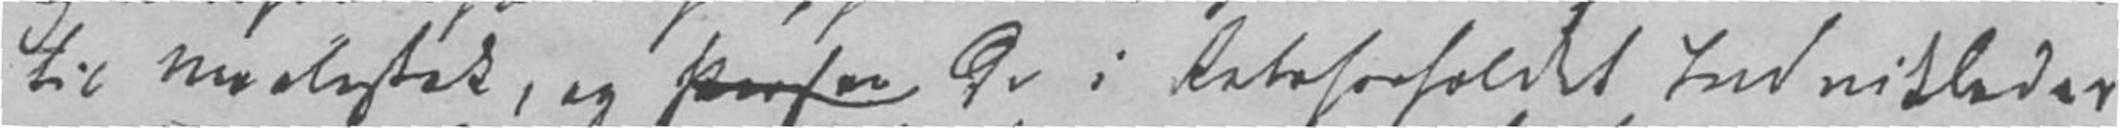til Maalestok, og person de i Retsforholdet Indvikledes
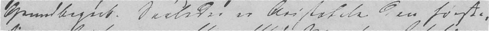Grundbegreb. Saaledes er Aristoteles den første,
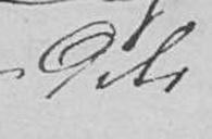dets
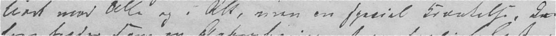Uret mod Alle og i Alt, men en speciel Krænkelse, der
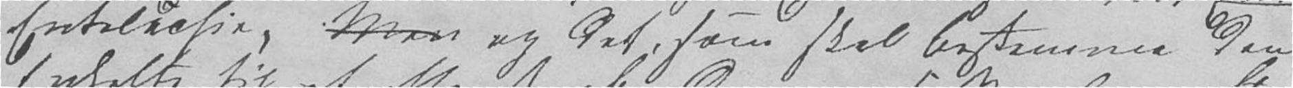Entelechio, Men og det, som skal bestemme den
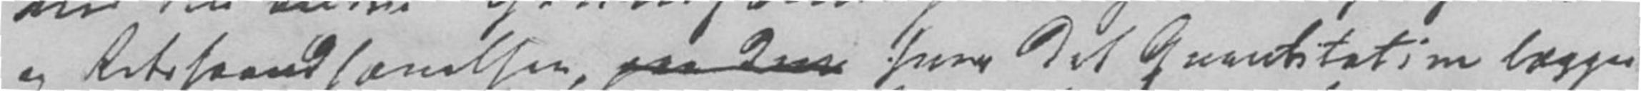og Retshaandsævelsen, paa dens hvor det Qvantitative lægges
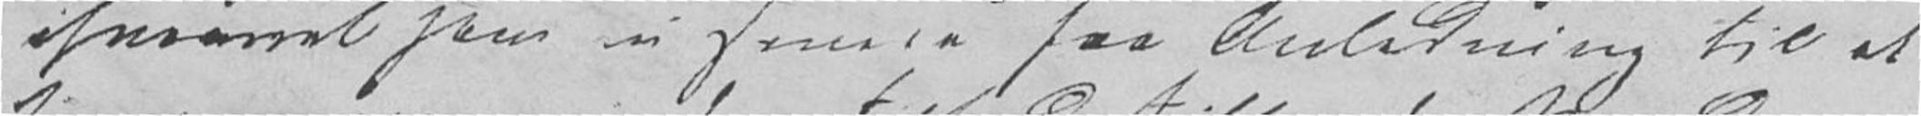ihvorvel som vi senere faa Anledning til at
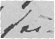saa-
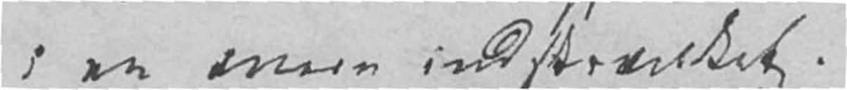i en mere indskrænket.
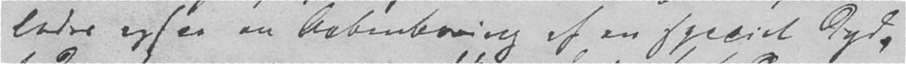ledes ogsaa en Aabenbaring af en speciel Dyd.
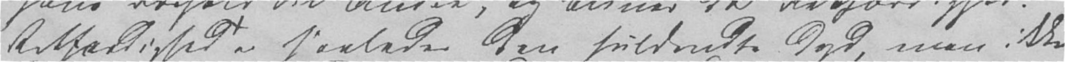Retfærdighed er saaledes den fuldendte Dyd, men ikke
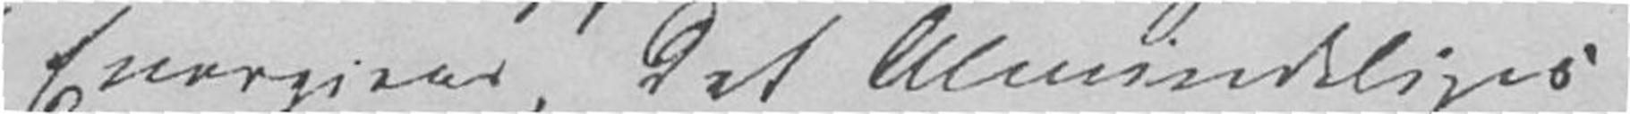Energiens, det Almindeliges
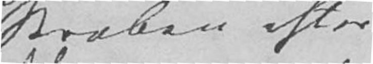Stræben efter
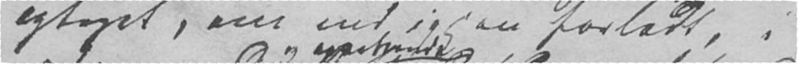optaget, om end igjen forladt, i
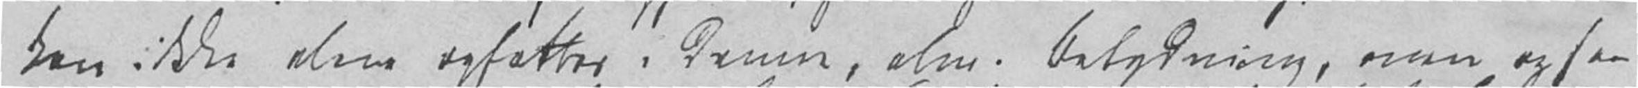kan ikke alene opfattes. Dennes, alm. Betydning, men ogsaa
